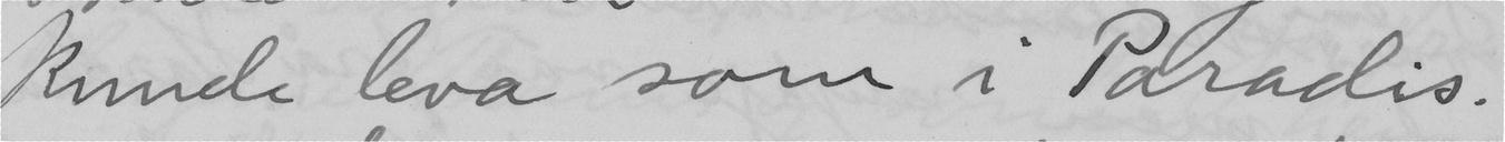kunde leva som i Paradis.
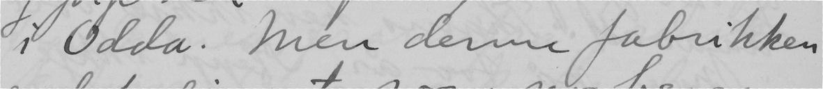i Odda. Men denne fabrikken
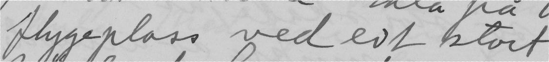flygeplass ved eit stort
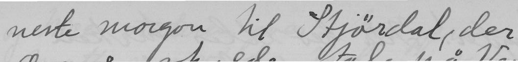neste morgon til Stjördal, der
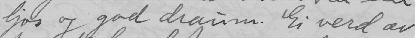ljos og god draum. Ei verd av
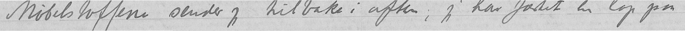Møbelstoffene sender jeg tilbake i aften; jeg har fæstet en lap paa
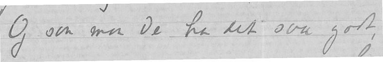Og saa maa De har det saa godt,
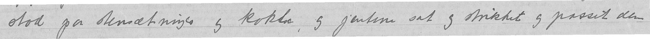stod paa stensætninger og kokte, og jentene sat og strikket og passet dem
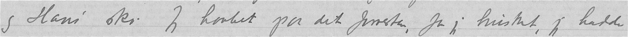og Hans' sko. Jeg haabet paa det forresten, for jeg husket, jeg hadde
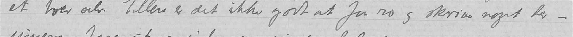et brev selv. Ellers er det ikke godt at faa ro og skrive noget her -
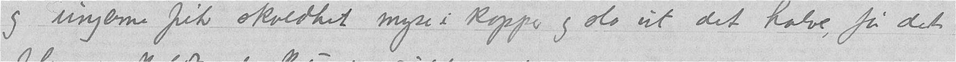og ungerne fik skoldhet myse i kopper og slo ut det halve, før det
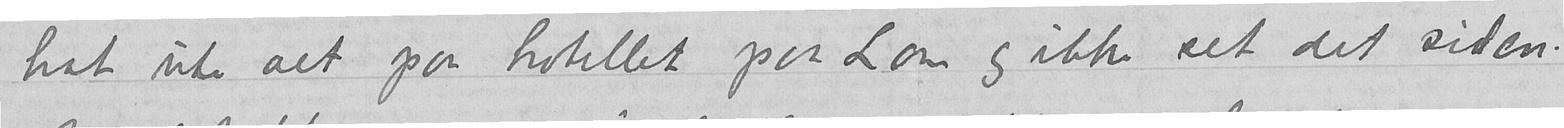hat ute alt paa hotellet paa Lom og ikke set det siden.
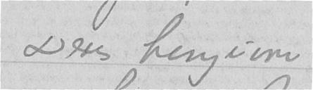Deres hengivne
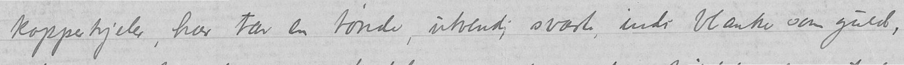kopperkjeler, hver tar en tønde, utvendig svarte, indi blanke som guld,
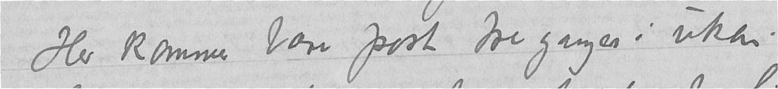Her kommer bare post tre ganger i uken.
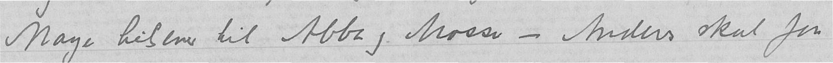Mange hilsener til Abba og Mosse – Anders skal faa
